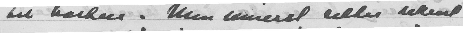til høsten. Men smeset sikter sikenet
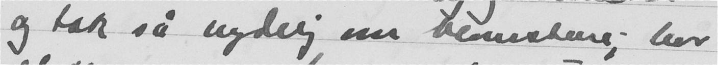og tok så nydelig om blomstene; har
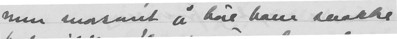men morsomt å høre han snakke
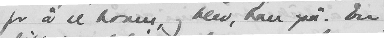for å se haven og blev, han også. En
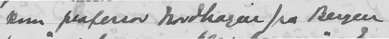kom professor Nordhagen fra Bergen
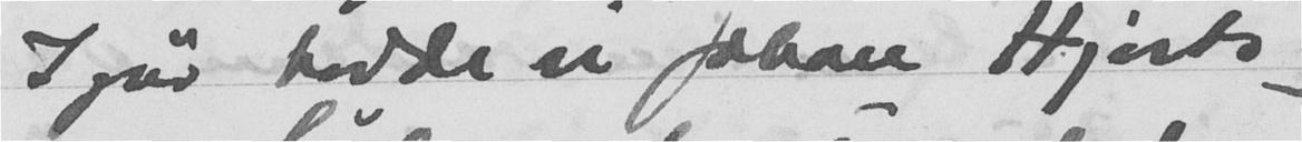Igår hadde vi Johan Hjorts og
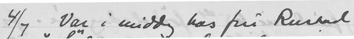4/7 Var i middag hos fru Rusbad
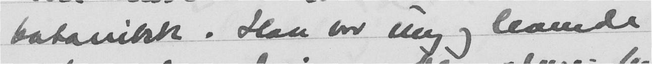botanikk. Han var mig og levende
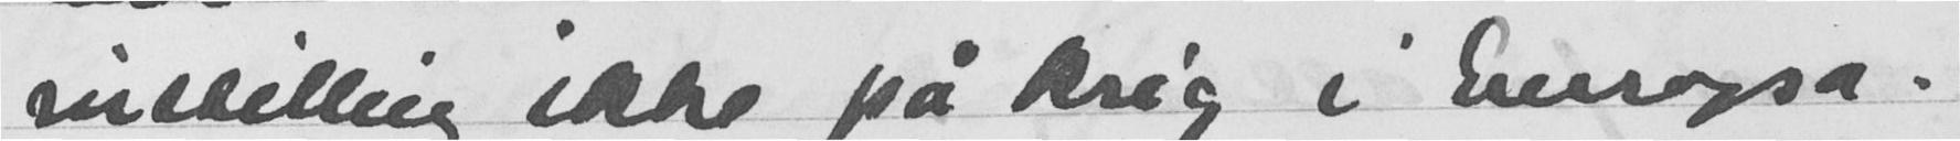innstilling ikke på krig i Europa.
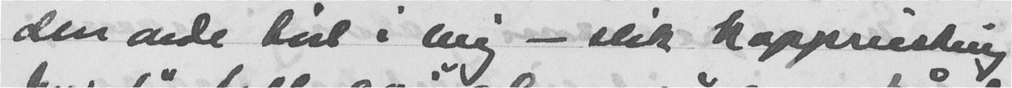den ande tvil i mig - slik kapprusting
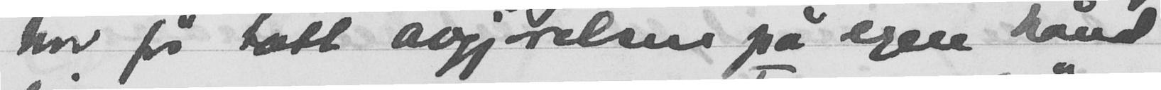har før tatt avgjørelsen på egen hånd
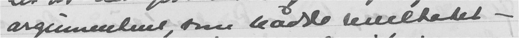argumentene, som nådde resultatet -
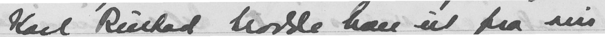Karl Rustad trodde han ut fra sin
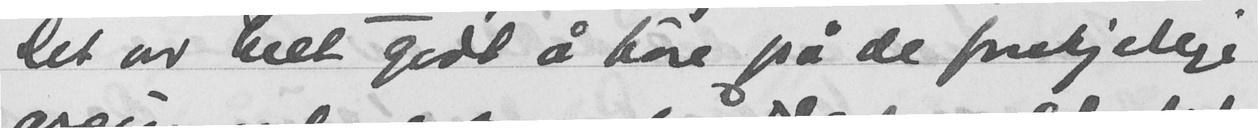Det var helt godt å høre på de forskjellige
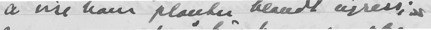å vise han planter blandt nyrestje
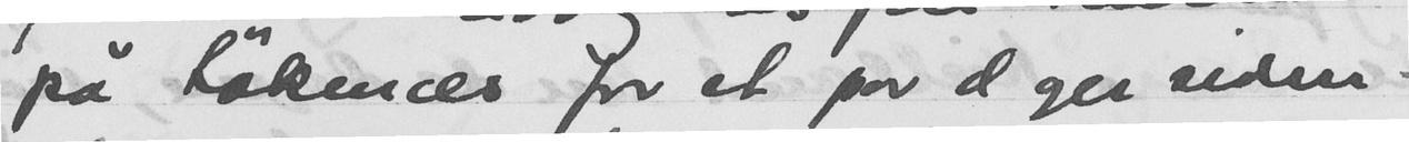på Løkenes for et par dager siden.
